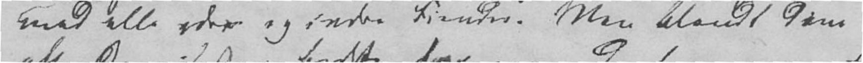mod alle ydre og indre Fiender. Men blandt dem
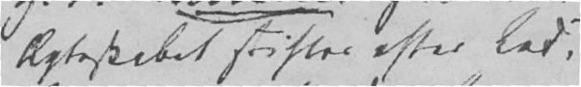Ægteskabet stiftes efter Lod,
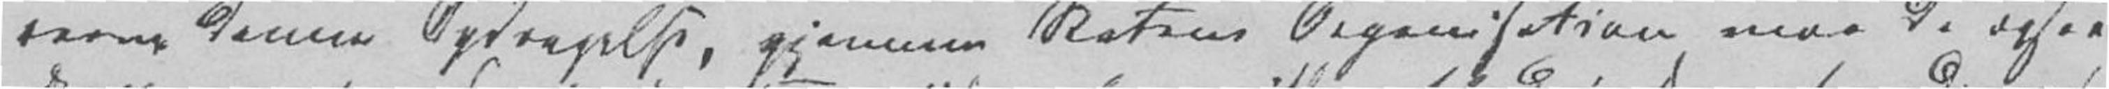rerne denne Opdragelse, gjennem Statens Oragnisation maa de ogsaa
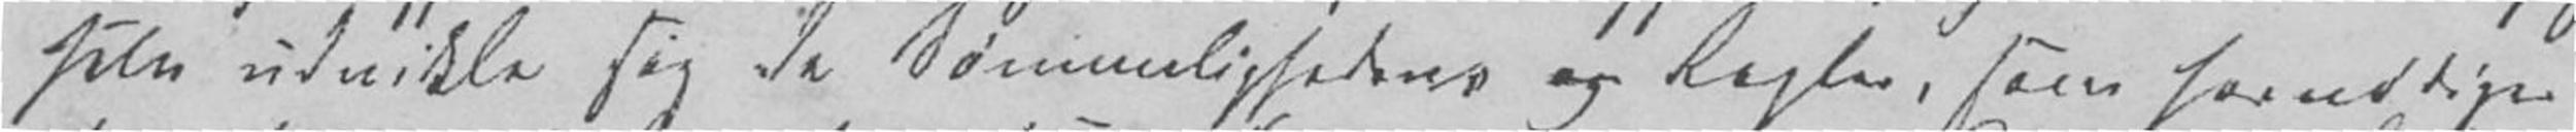selv udvikle sig da Sømmelighedens og Regler, som fornødigen
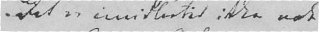Det er imidlertid ikke nok
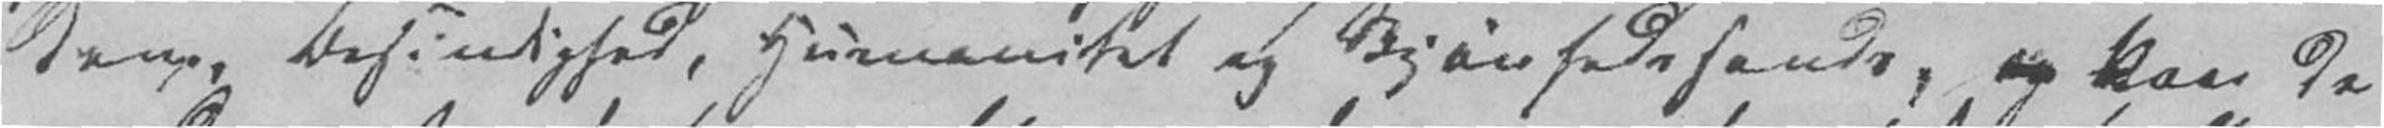dom, Besindighed, Humanitet og Skjønhedssands, og Naar de
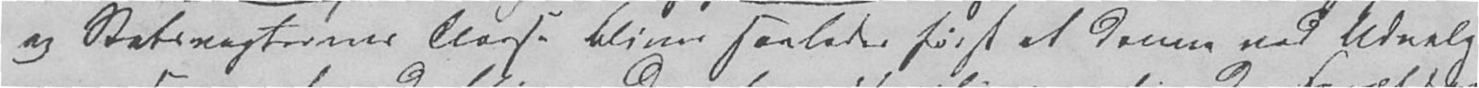og Statsmagternes Classe bliver saaledes først at danne ved Udvalg
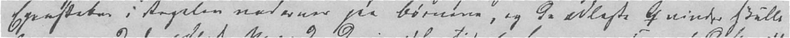Egenskaber i Regelen nedarver paa Børnene, og de ædleste Qvinder skulle
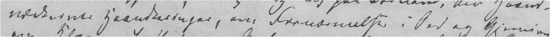værkernes Haandskæringer, om Fornærmelse i Ord og Gjerning,
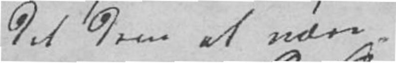det dem at være
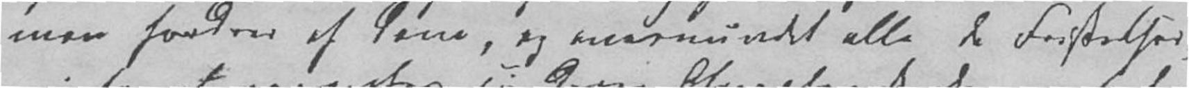man fordrer af dem, og overvundet alle de Fristelser,
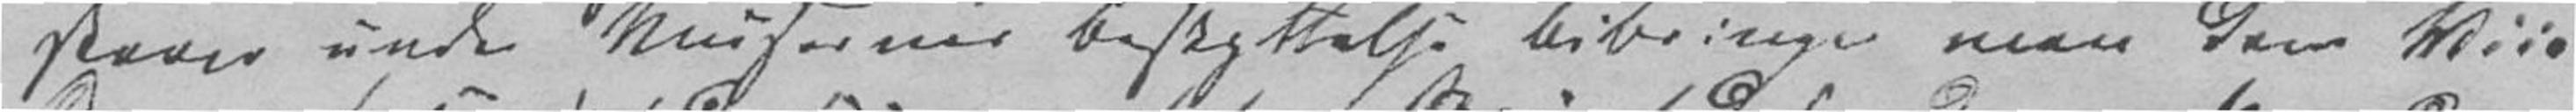staaer under Musernes Beskyttelse bibringer man dem Viis-
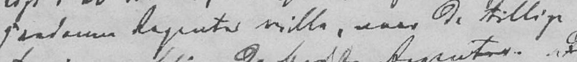saadanne Regenter ville, naar de tillige
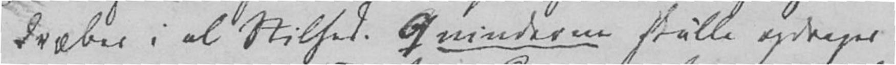dræbes i al Stilhed. Qvinderne skulle opdrages
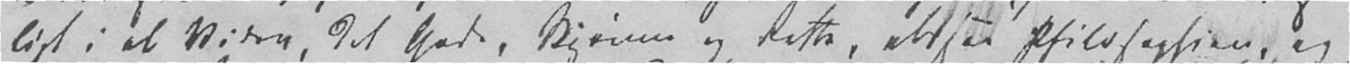ligt i al Viden, det Gode, Skjønne og Rette, altsaa Philosophien, og
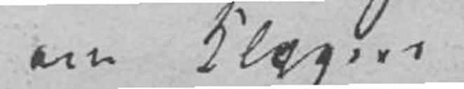om Klagers
